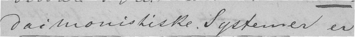daimonistiske Systemer er
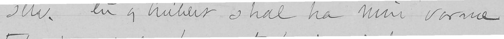selv. Du og Aubert skal ha min varme
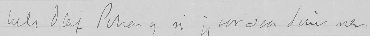hils Olaf Schou og si jeg var saa dum ner-
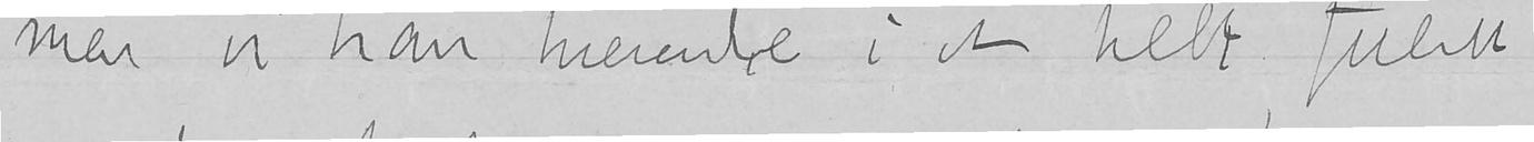men vi har hverandre i et helt fuldt
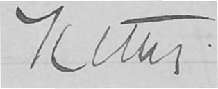Kitty.
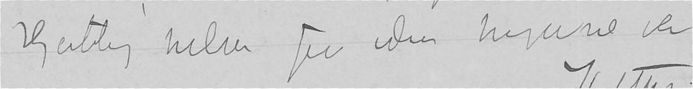Hjertelig hilsen fra eders hengivne ven
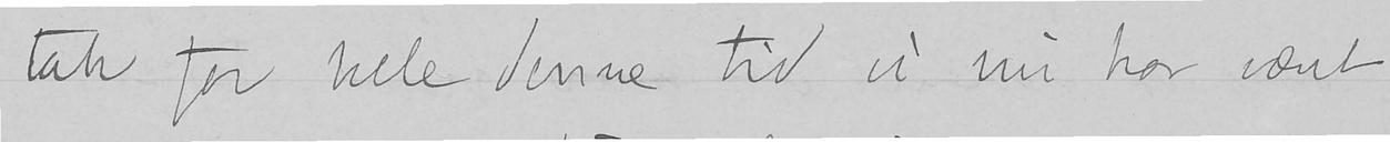tak for hele denne tid vi nu har været
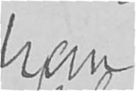han
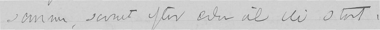sammen, savnet efter eder vil bli stort,
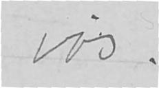vøs.
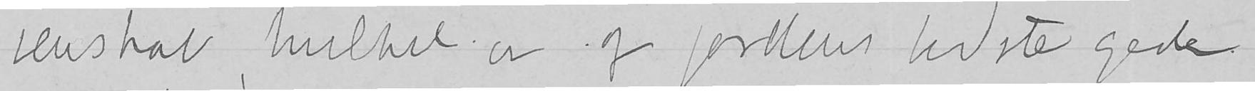venskab, hvilket er og forbliver bedste gode.
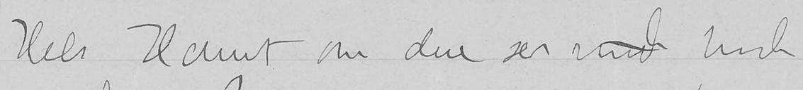Hils Harriet om dere ser hend hende
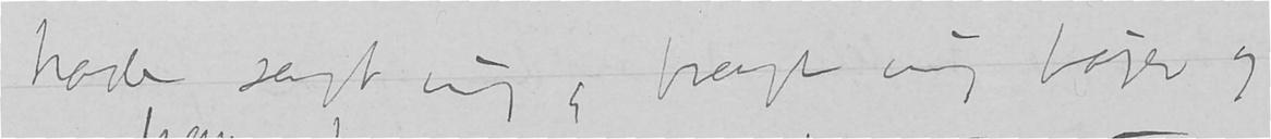havde sagt mig, bragt mig bøger og
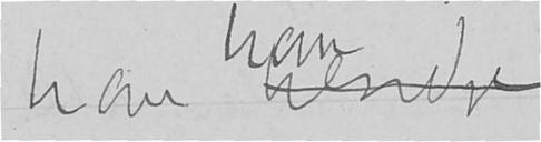han brendge
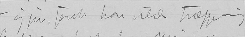igjen, fordi han vilde træffe mig
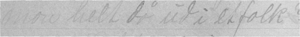mon helt dø ud i et folk?
